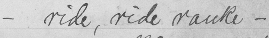- ride, ride ranke -
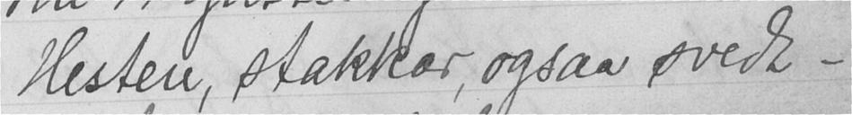Hesten, stakkar, ogsaa svedt -
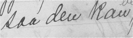saa den kan
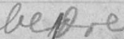bedre
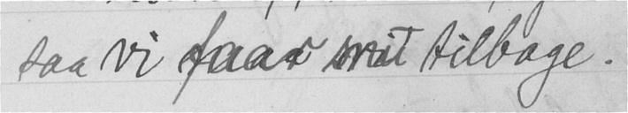saa vi faar snu tilbage.
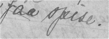faa spise.
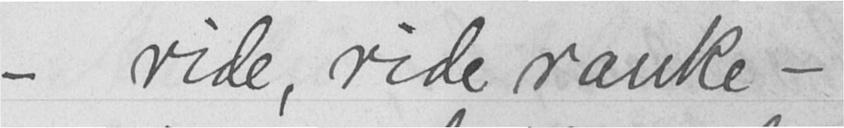- ride, ride ranke -
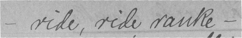- ride, ride ranke -
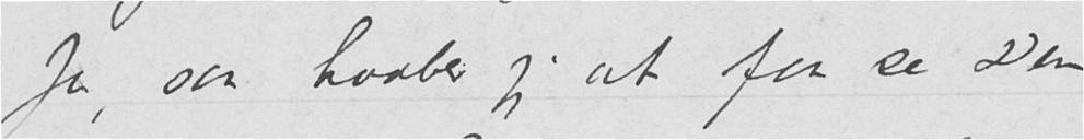Ja, saa haaber jeg at faa se Dem
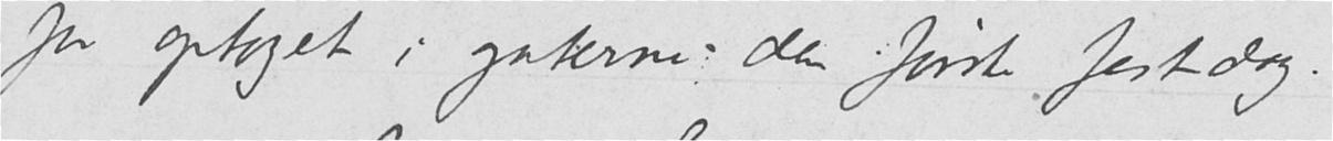for optoget i gaterne - den første festdag.
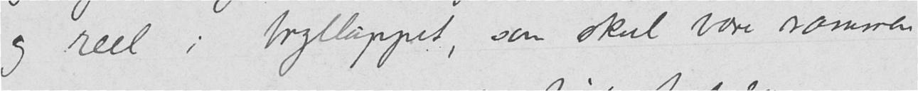og reel i brylluppet, som skal være rammen
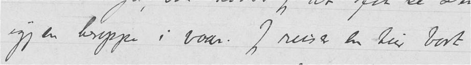igjen heroppe i vaar. Jeg reiser en tur bort
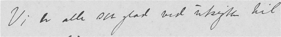Vi er alle saa glad ved utsigten til.
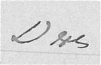Deres
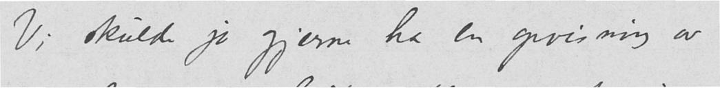Vi skulde jo gjerne ha en opvisning av
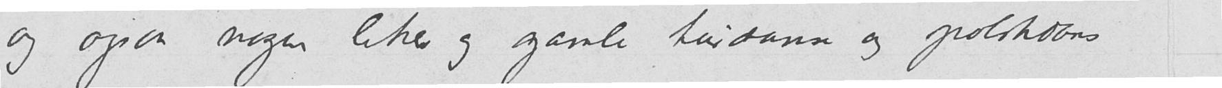og ogsaa nogen like og gamle turdanse og polskdans
